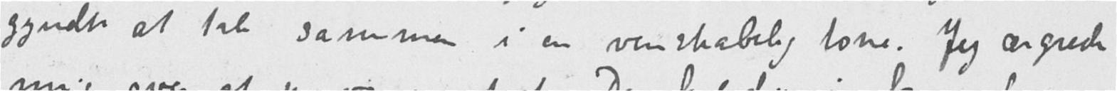gyndte at tale sammen i en venskabelig tone. Jeg ærgrede
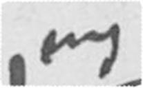mig
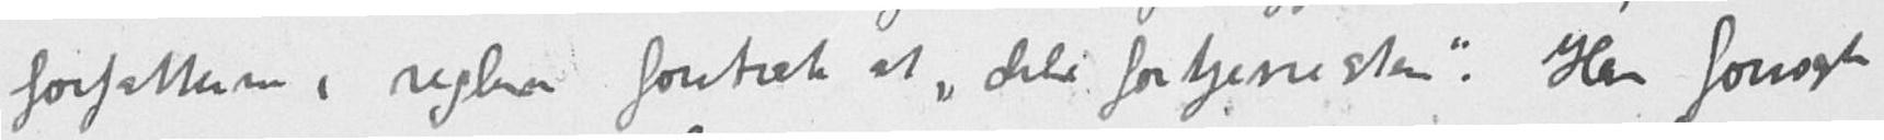forfatterne i reglen foretrak at "dele fortjenesten". Han forsøgte
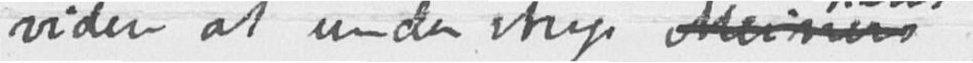videre at understrege Meiners
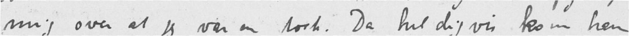mig over at jeg var en tosk. Da heldigvis kom han
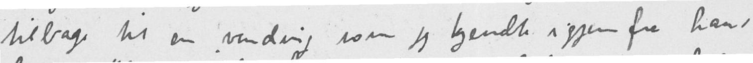tilbage til en vending som jeg kjendte igjen fra hans
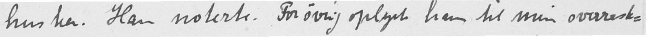husker. Han noterte. Forøvrig oplyste han til min overrask-
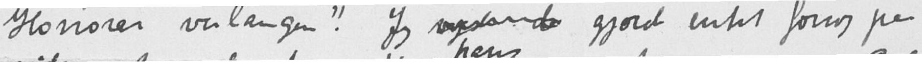Honorar verlangen". Jeg sagde da gjorde intet forsøg paa
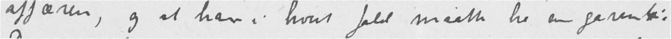affæren, og at han i hvert fald maatte ha en garanti.
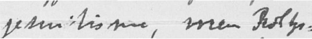jesuitisme, men Boltz-
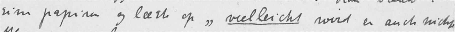sine papirer og læste op "villeicht wird er auch nicht
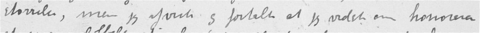størrelse, men jeg afviste og fortalte at jeg vidste om honorarer
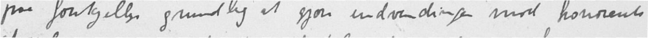paa forskjellige grundlag at gjøre indvendinger mod honorarets
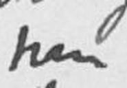han
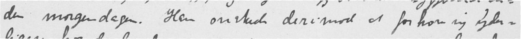den morgendagen. Han ønskede derimod at forhøre sig yder-
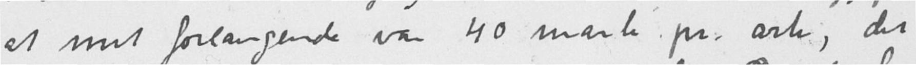at mit forlangende var 40 mark pr. ark, det
